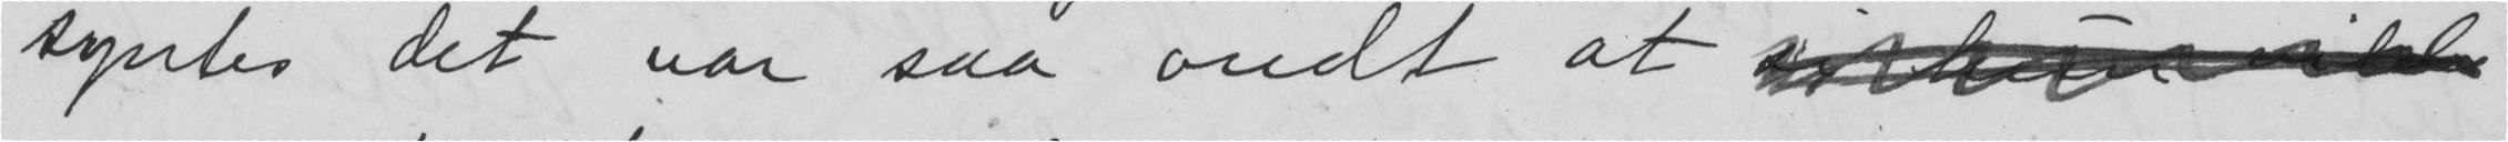syntes det var saa ondt at
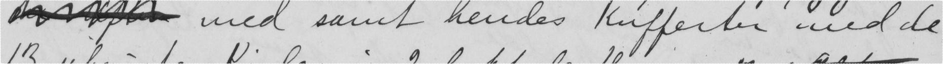med samt hendes Kufferter med de
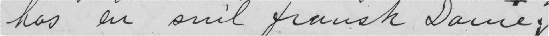hos en snil fransk Dame
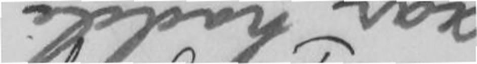aar hadde
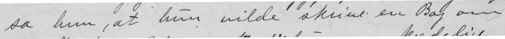sa hun, at hun vilde skrive en Bog om
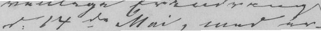d. 14de Mai, med er-
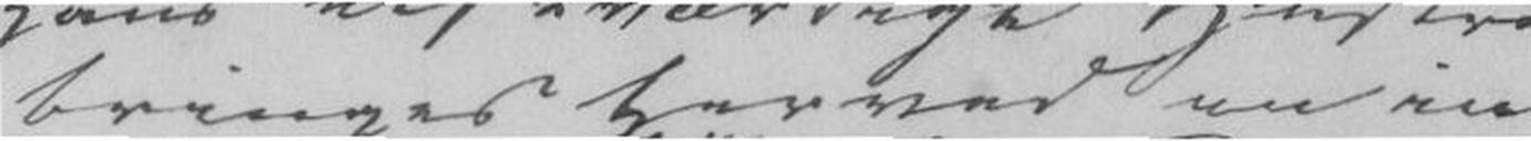bringes herved en in-
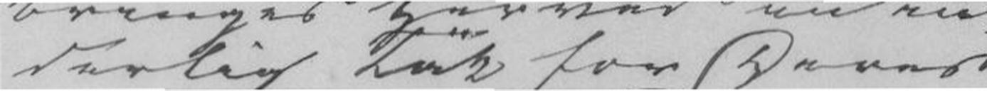derlig Tak for Deres
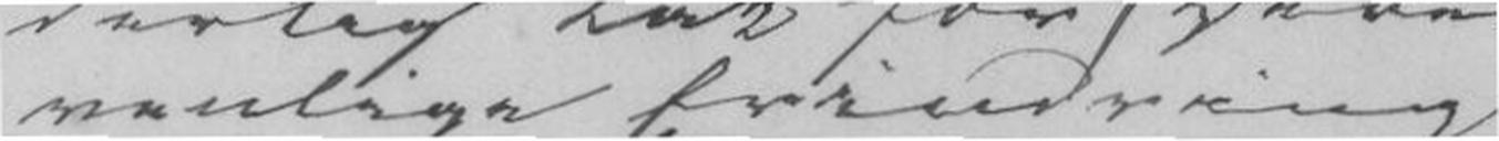venlige Erindring
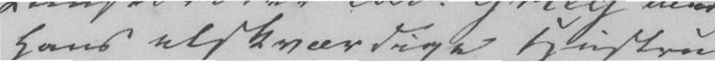hans elskværdige Hustru
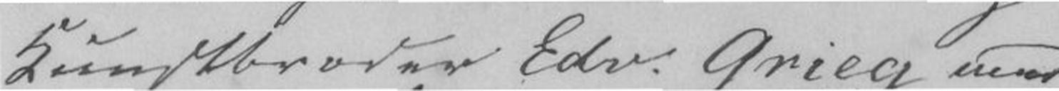Kunstbroder Edv. Grieg med
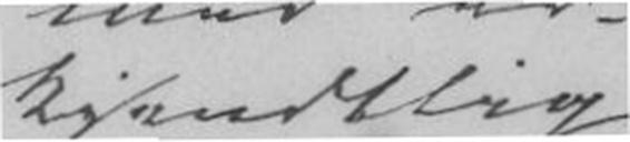kjendtlig
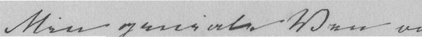Min geniale Ven og
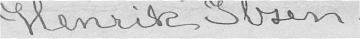Henrik Ibsen.
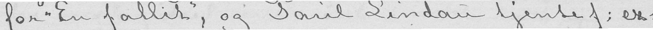for «En fallit», og Paul Lindau tjente f; ex:
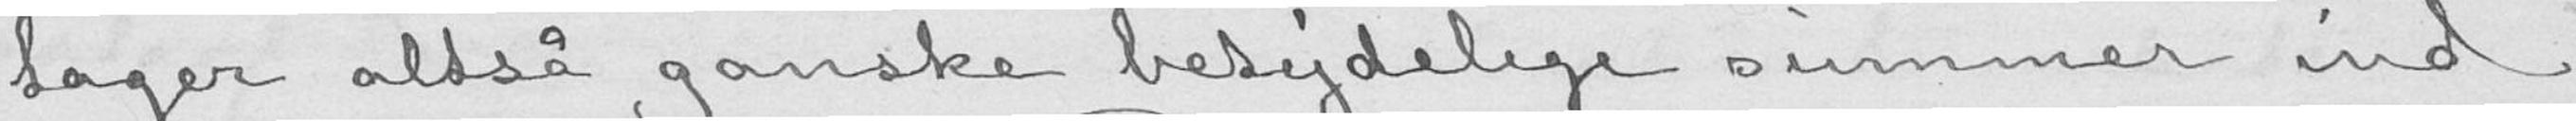tager altså ganske betydelige summer ind
